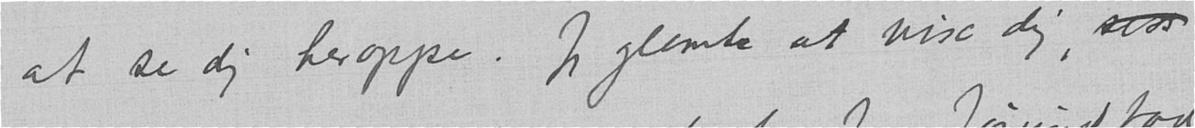at se dig heroppe. Jeg glemte at vise dig, siss
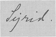Sigrid.
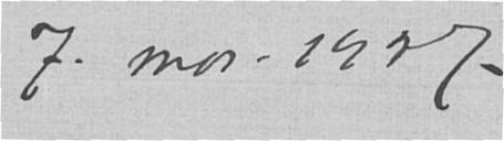7. mai - 1927.
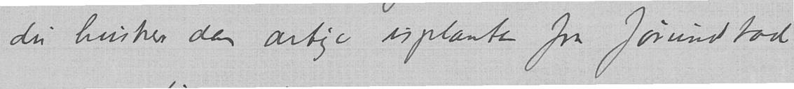du husker den artige isplanten fra Jørundbad
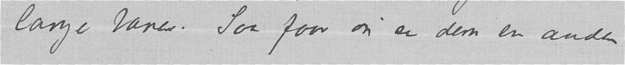lange baner. Saa faar du se dem en anden
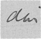din
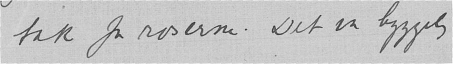tak for roserne. Det var hyggelig
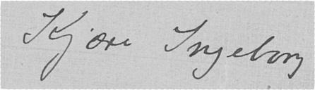Kjære Ingeborg
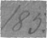183.
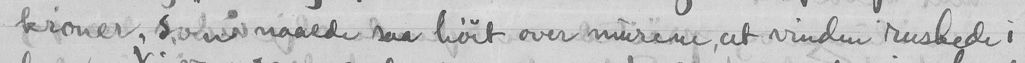kroner, som naaede, saa høit over murene, at vinden ruskede i
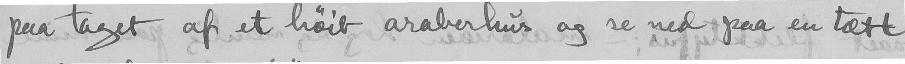paa taget af et høit araberhus, og se ned paa en tætt
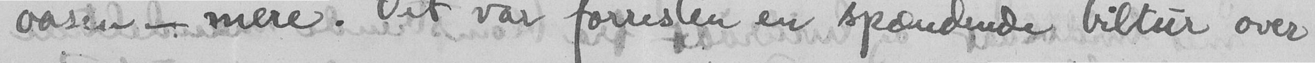oasen - mere. Det var forresten en spændende biltur over
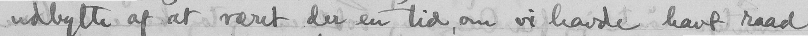udbytte af at været der en tid, om vi havde havt raad
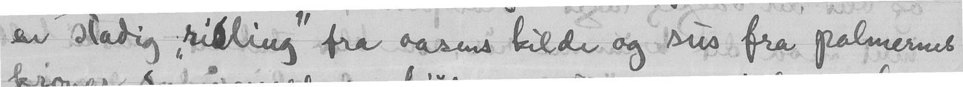en stadig "ridling" fra oasens kilde og sus, fra palmernes
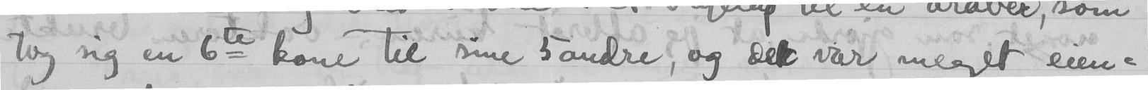tog sig en 6te kone til sine 5 andre, og det var meget eien-
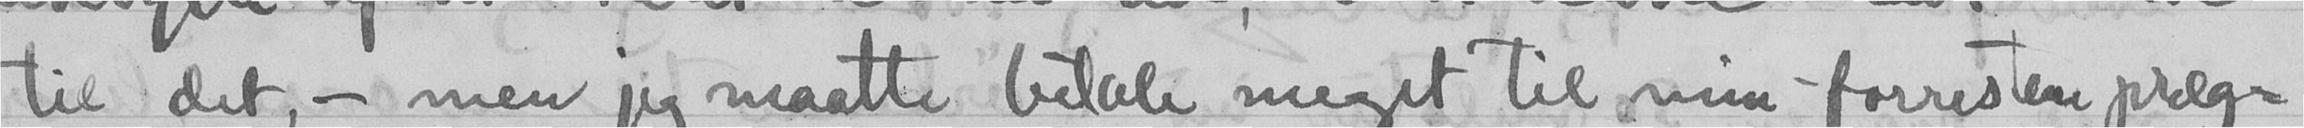til det, - men jeg maate betale meget til min forresten præg-
